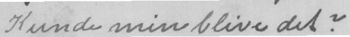Kunde min blive det? - - -
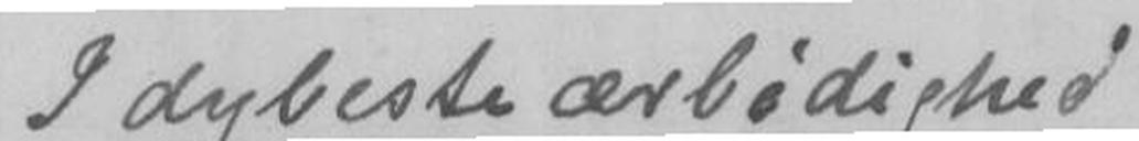I dybeste ærbødighed
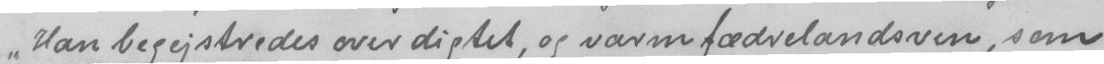Han begejstredes over digtet, og varm fædrelandsven, som
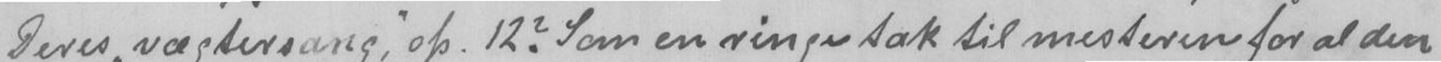Deres vægtersang, op. 122 Som en ringe tak til mesteren for al den
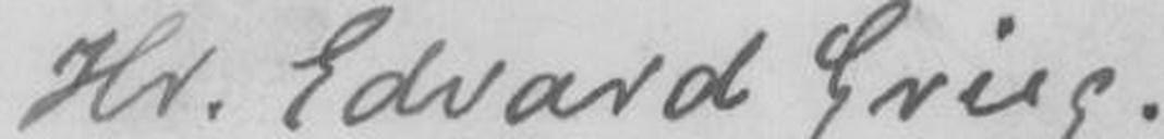Hr. Edvard Grieg.
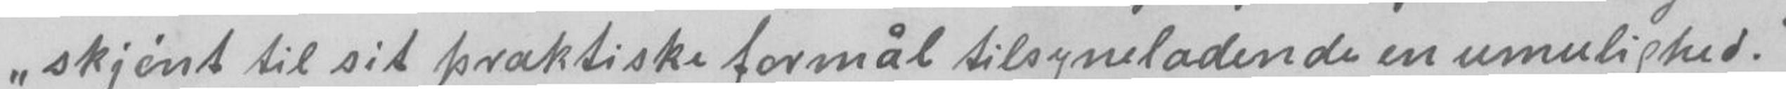skjønt til sit praktiske formål tilsyneladende en umulighed.
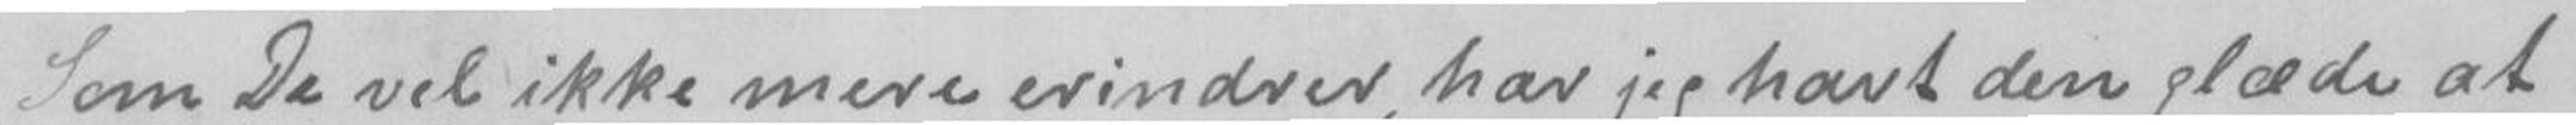Som De vel ikke mere erindrer, har jeg havt den glæde at
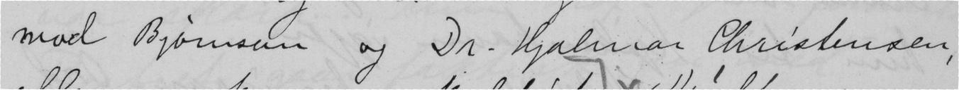mod Bjørnson og Dr. Hjalmar Christensen,
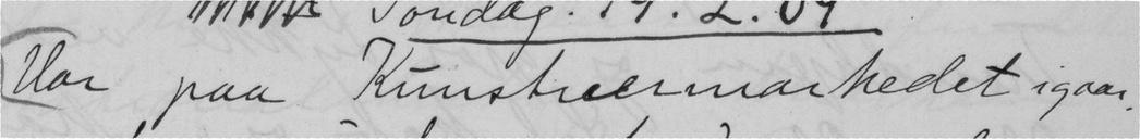Var paa Kunstnermarkedet igaar.
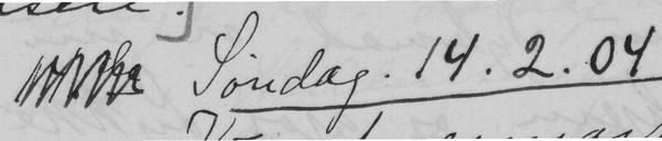Søndag. 14.2.04
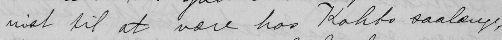vist til at være hos Kohts saalænge,
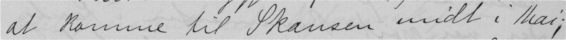at komme til Skansen midt i Mai;
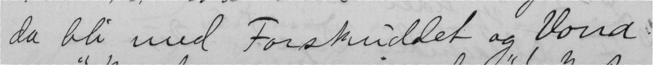da bli med Forskuddet og Vona.
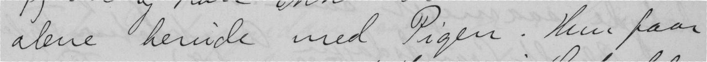alene herude med Pigen. Hun faar
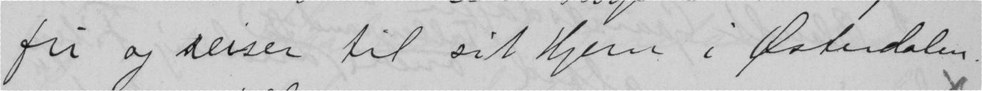fri og reiser til sit Hjem i Østerdalen.
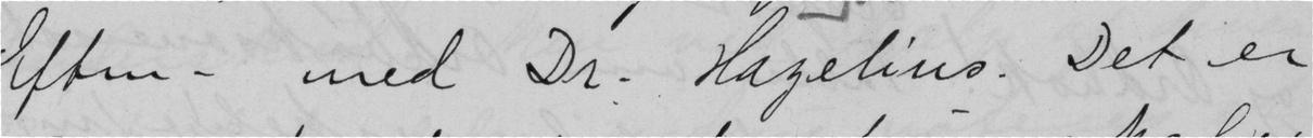Eftm - med Dr. Hagelius. Det er
